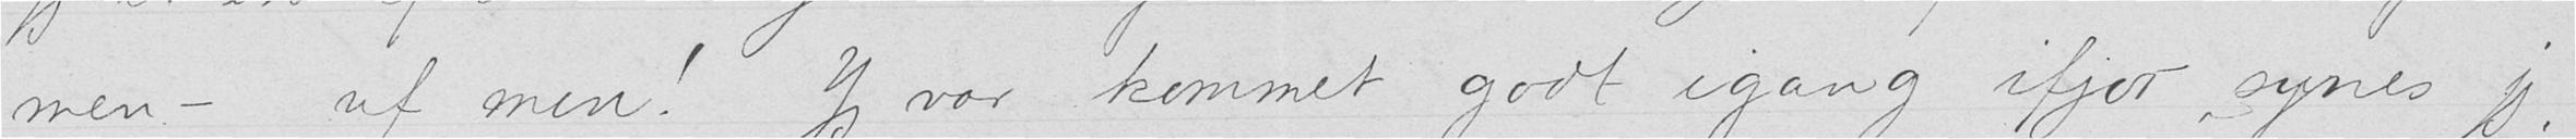men - uf men! Jeg var kommet godt igang ifjor, synes jeg,
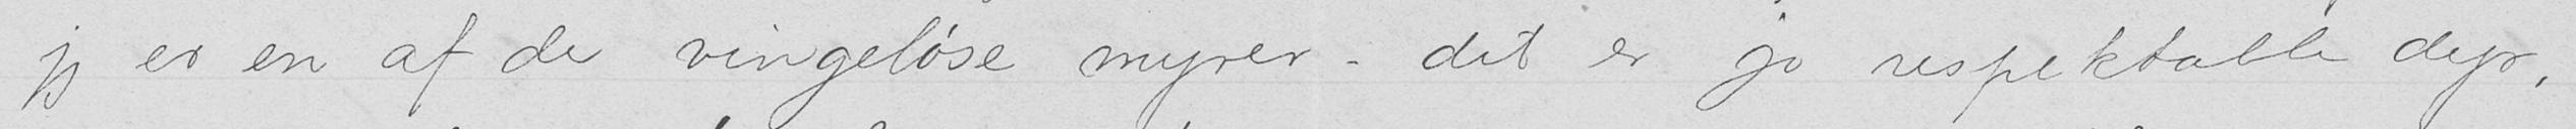jeg er en af de vingeløse myrer -  det er jo respektable dyr,
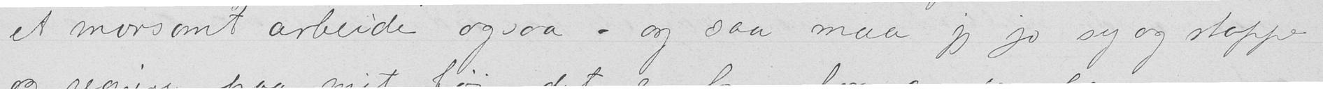et morsomt arbeide ogsaa - og saa maa jeg jo sy og stoppe
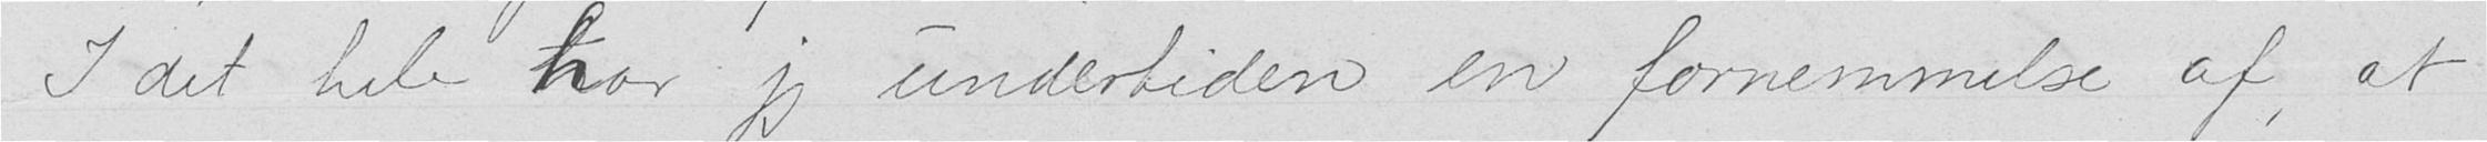I det hele har jeg undertiden en fornemmelse af, at
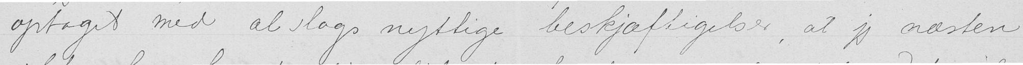optaget med al slags nyttige beskjæftigelser, at jeg næsten
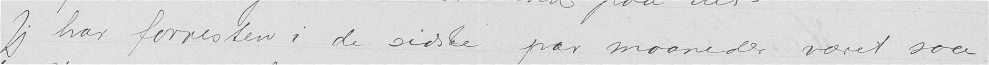Jeg har forresten i de sidste par maaneder været saa
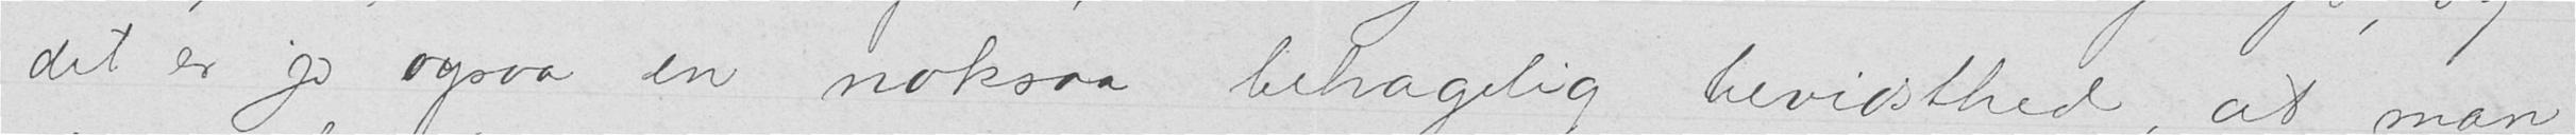det er jo ogsaa en noksaa behagelig bevidsthed, at man
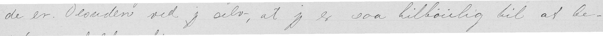de er. Desuden ved jeg selv, at jeg er saa tilbøilig til at be-
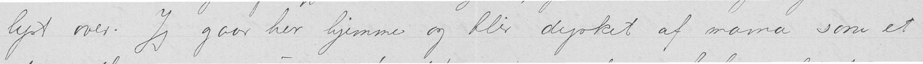lyst over. Jeg gaar her hjemme og blir dyrket af mama som et
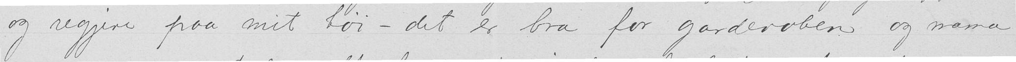og regjere paa mit tøi - det er bra for garderoben og mama
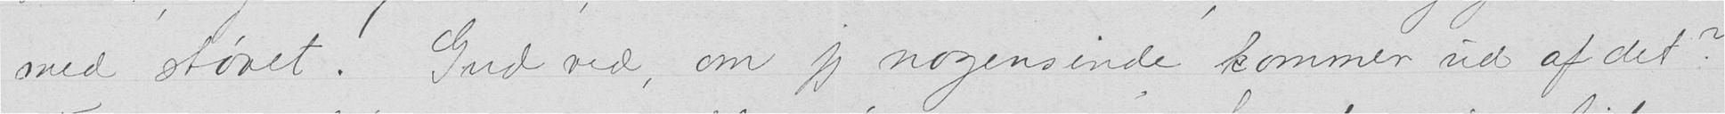med støvet. Gudved, om jeg nogensinde kommer ud af det?
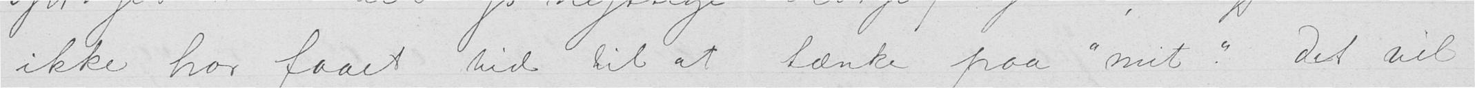ikke har faaet tid til at tænke paa "mit". Det vil
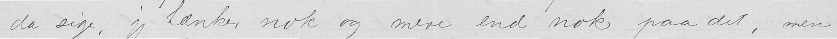da sige, jeg tænker nok og mere end nok paa det, men
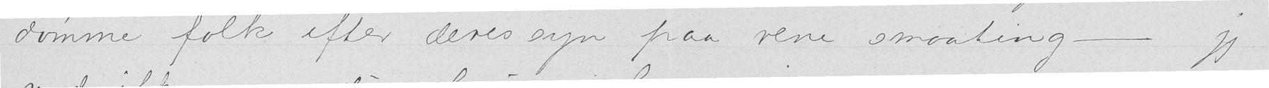dømme folk efter deres syn paa rene smaating - jeg
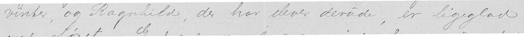vinter, og Ragnhild, der har elever derude, er ligeglad
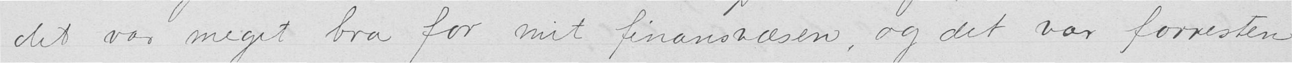det var meget bra for mit finansvæsen, og det var forresten
